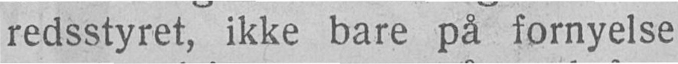redsstyret, ikke bare på fornyelse
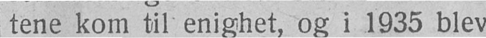tene kom til enighet, og i 1935 blev
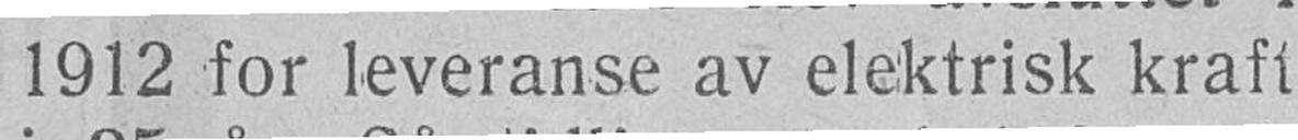1912 for leveranse av elektrisk kraft
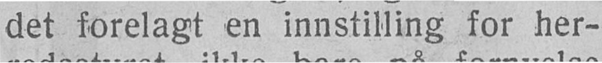det forelagt en innstilling for her-
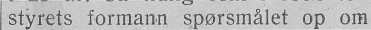styrets formann spørsmålet op om
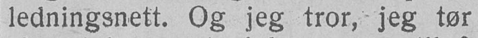ledningsnett. Og jeg tror, jeg tør
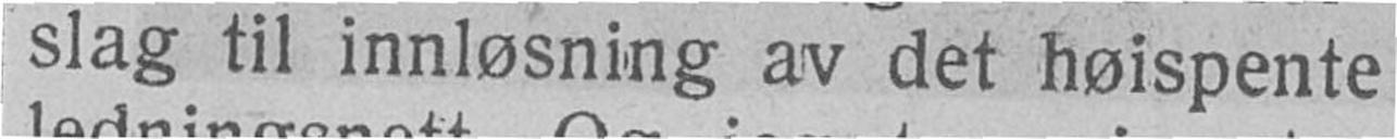slag til innløsning av det høispente
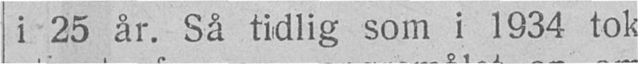i 25 år. Så tidlig som i 1934 tok
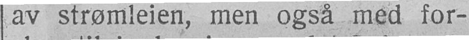av strømleien, men ogsâ med for-
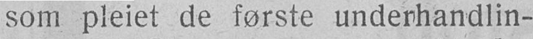som pleiet de første underhandlin-
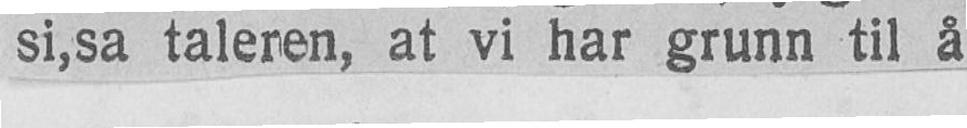si,sa taleren, at vi har grunn til å
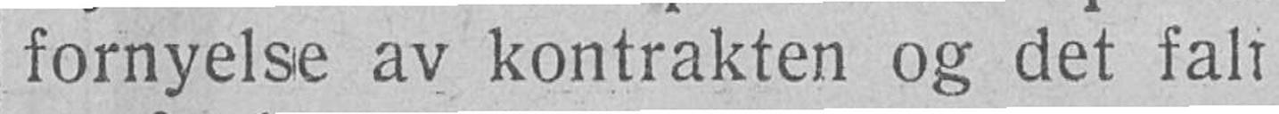fornyelse av kontrakten og det falt
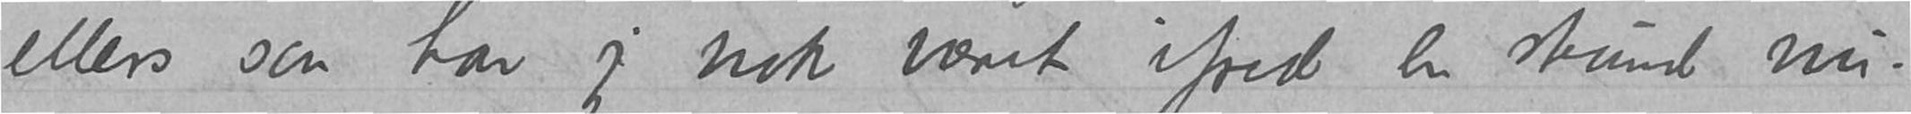ellers saa har jeg nok været ifred en stund nu.
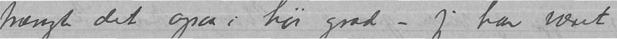Jeg trængte det ogsaa i høi grad – jeg har været
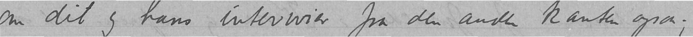om dit og hans interwier fra den anden kanten ogsaa;
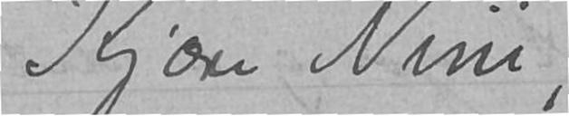Kjære Nini
